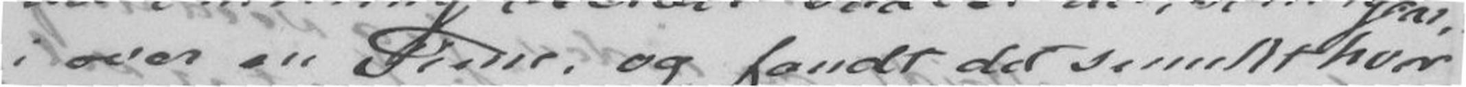i over en Time, og fandt det smukt hvor
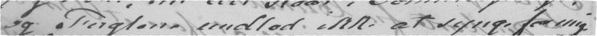og Fuglene undlod ikke at synge for mig.
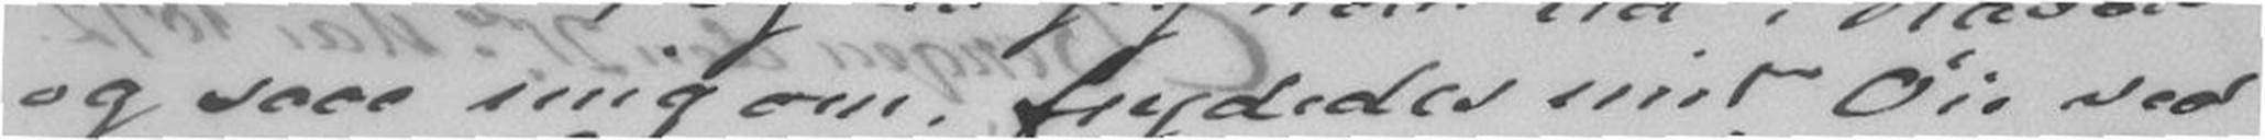og seer mig om, frydedes mit Øie ved
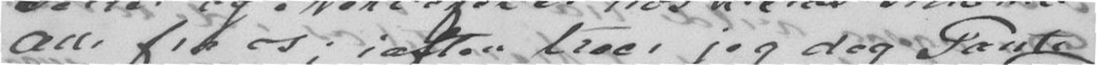Alle fra os; iaften troer jeg dog Tante
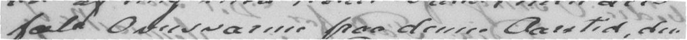fæle Ovnsvarme paa denne Aarstid, den
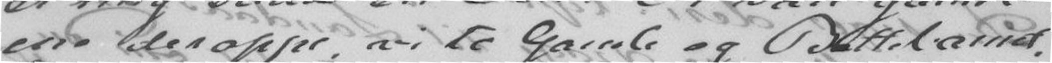ene deroppe, vi to Gamle og Bettebarnet.
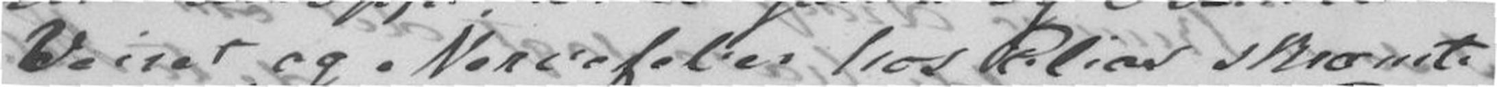Veiret og Nervefeber hos Elias skræmte
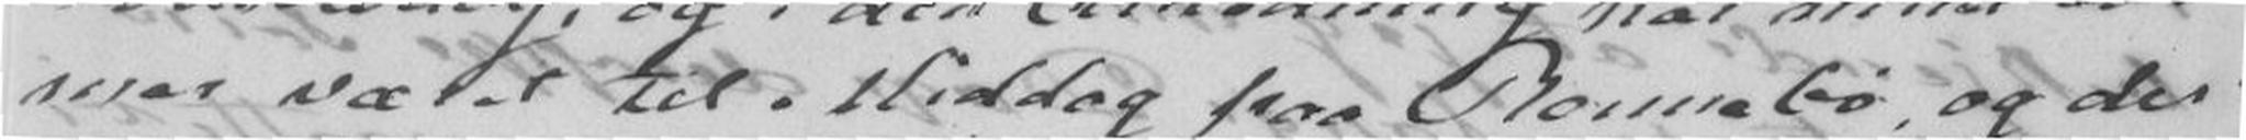mer været til Middag paa Rennebø, og der
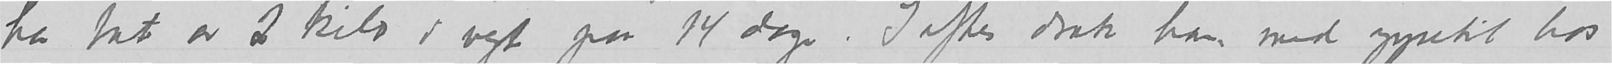har tat av 2 kilo i vegt paa 14 dager. I aftes  drak han med appetit hos
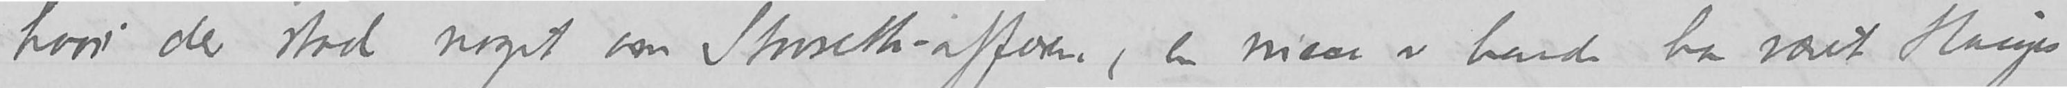hvori der stod noget om Stavenseth – affæren (en niese av hende har været Hauges
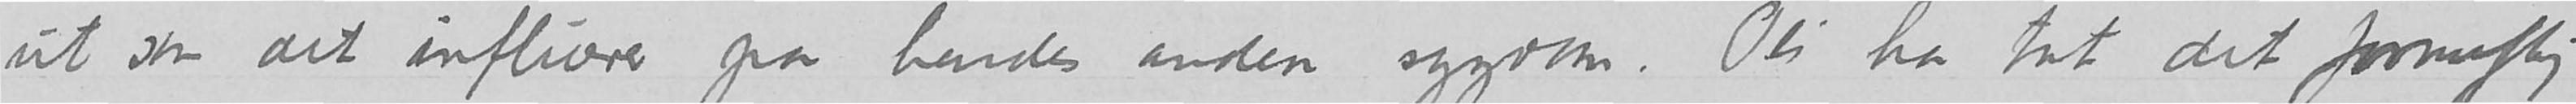ut som det influerer paa hendes anden sygdom. Pei har tat det fornuftig
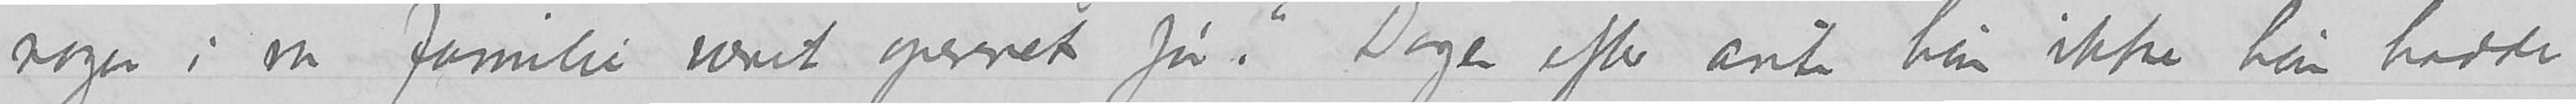nogen i vaar familie været opereret før.»  Dagen efter ante hun ikke hun hadde
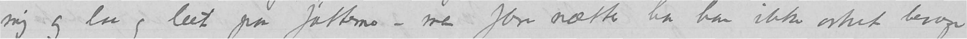mig og laa og leet paa føtterne – men flere nætter har han ikke orket bevege
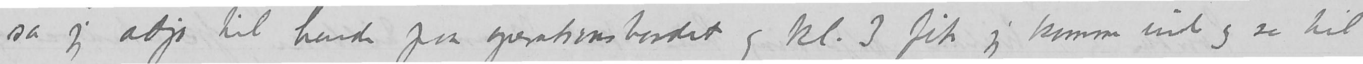sa jeg adjø til hende paa operationsbordet og kl. 3 fik jeg komme ind og se til
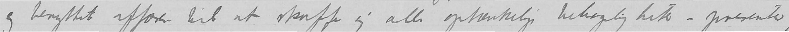og benyttet affæren til at skaffe sig alle opptænkelige behageligheter – presenter,
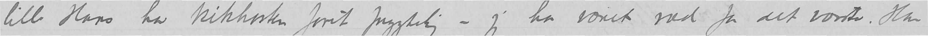lille Hans har kikhosten faret frygtelig – jeg har været ræd for det værste. Han
